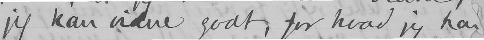jeg kan vidne godt, for hvad jeg har
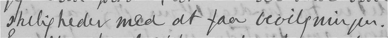skeligheder med at faa bevilgningen.
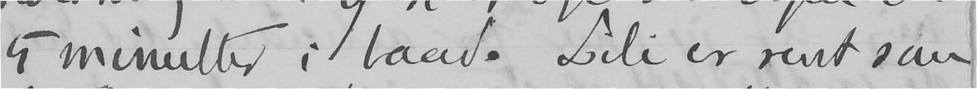5 minutter i baad. Lili er rent som
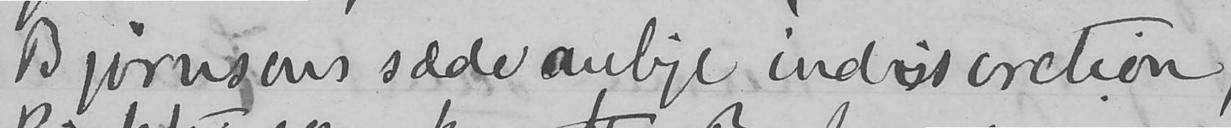Bjørnsons sædvanlige indiscretion,
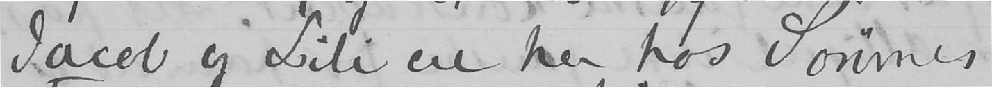Jacob og Lili ere her hos Sømmes
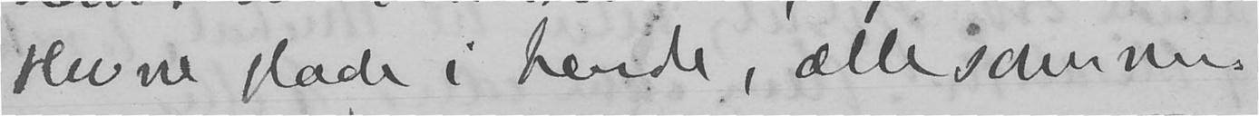blevne glade i hende, alle sammen.
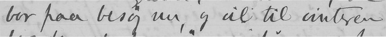bor paa besøg nu, og vil til vinteren
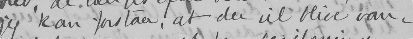jeg kan forstaa, at der vil blive van-
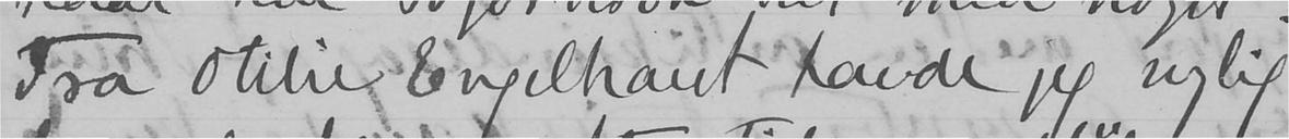Fra Otilie Engelhardt havde jeg nylig
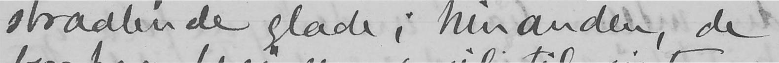straalende glade i hinanden, de
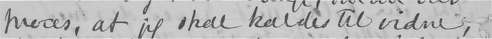proces, at jeg skal kaldes til vidne,
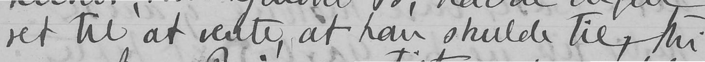ret til at vente, at han skulde tie, thi
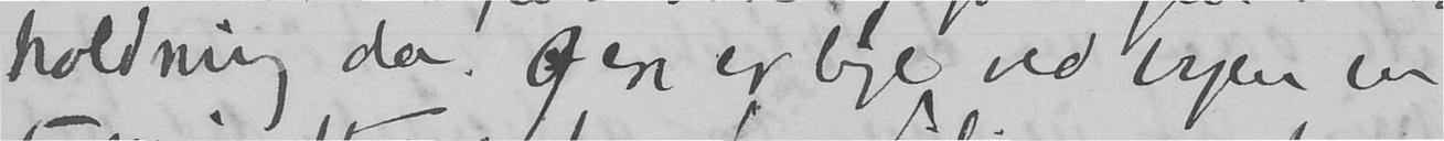holdning da. Øen er lige ved byen en
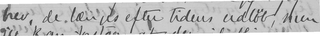brev, de længes efter tidens udløb, men
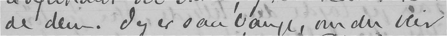de dem. Jeg er saa bange, om der blir
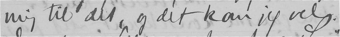ning til det, og det kan jeg vel.
